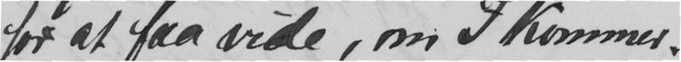for at faa vide, om I Kommer.
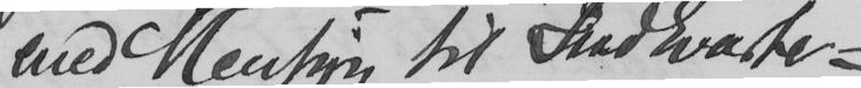med Hensyn til Indkvate-
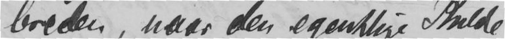breden, naar den egentlige Kulde
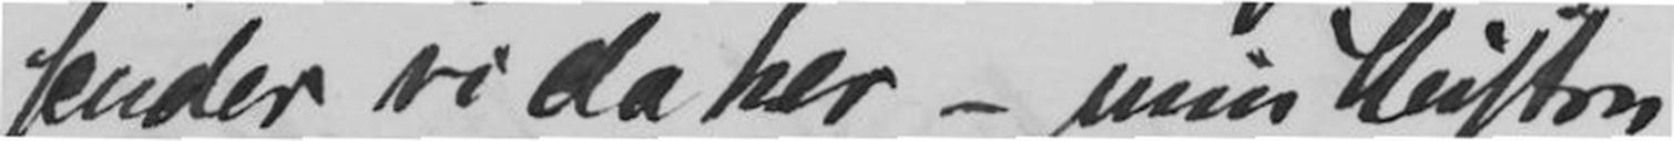sender vi da her - min Hustru
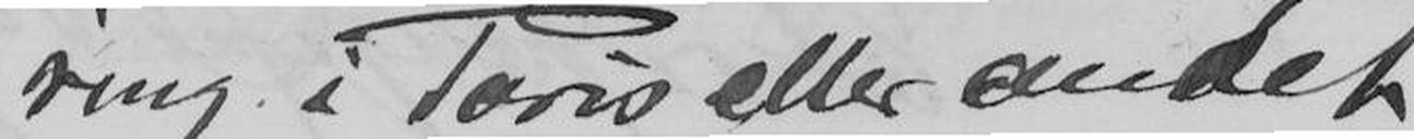ring i Paris eller andet,
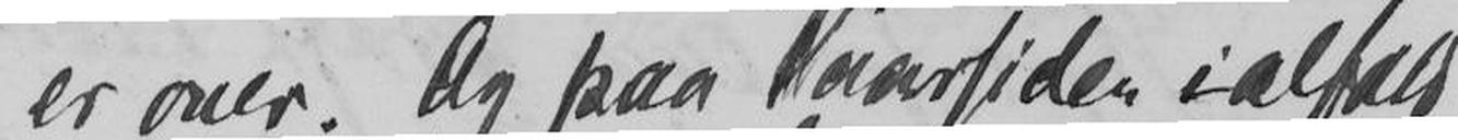er over. Og paa Vaarsiden ialfald
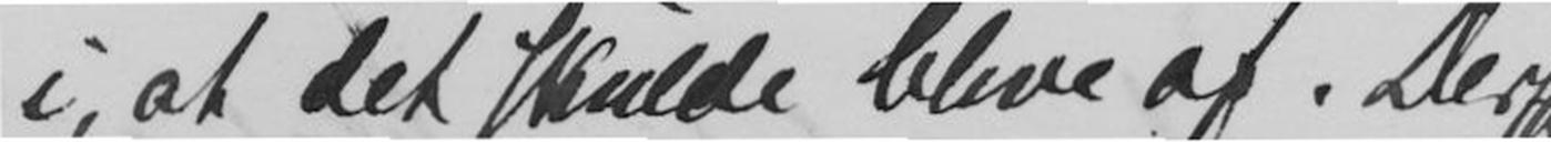i, at det skulde blive af. Derfor
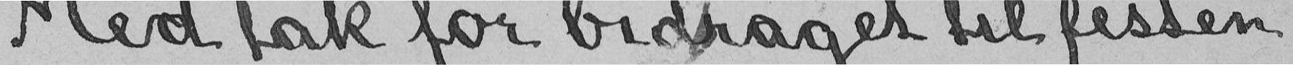Med tak for bidraget til festen
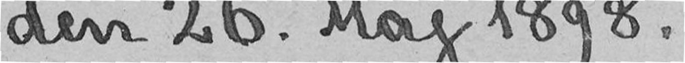den 26. Maj 1898.
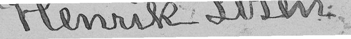Henrik Ibsen
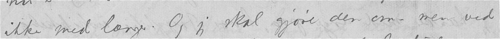ikke med længer. Og jeg skal gjøre den om - men ved
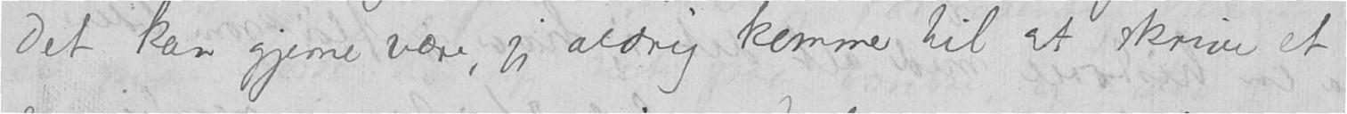Det kan gjerne være, jeg aldrig kommer til at skrive et
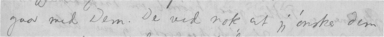gaar med Dem. De ved nok at jeg ønsker Dem
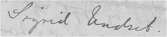Sigrid Undset.
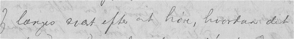Jeg længes svært efter at høre, hvordan det
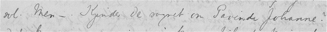sol. Men -. Kjender De sagnet om Pavinde Johanne?
